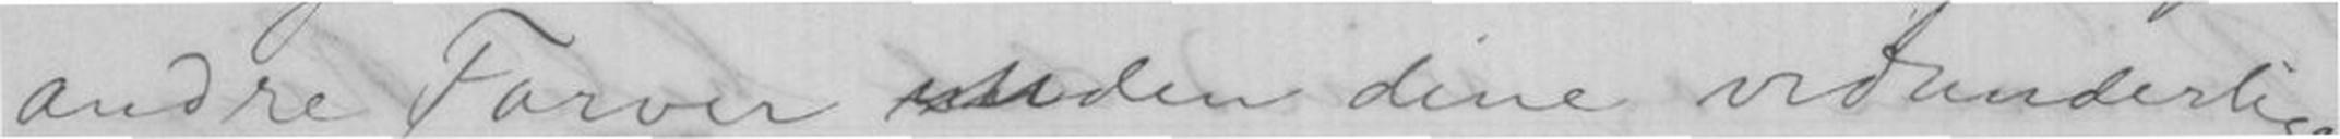andre Farver uden dine vidunderlige
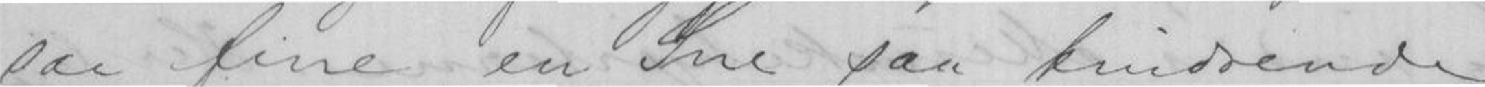saa fine, en Sne saa tindrende
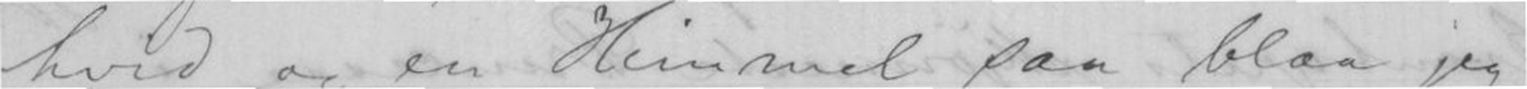hvid og en Himmel saa blaa jeg
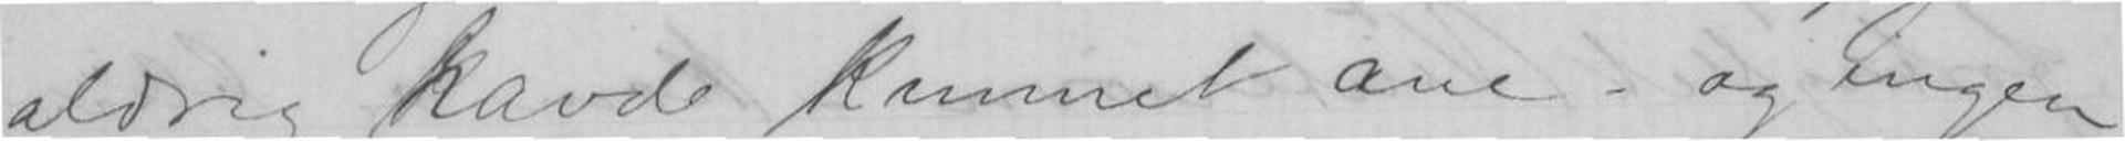aldrig havde kunnet ane - og ingen
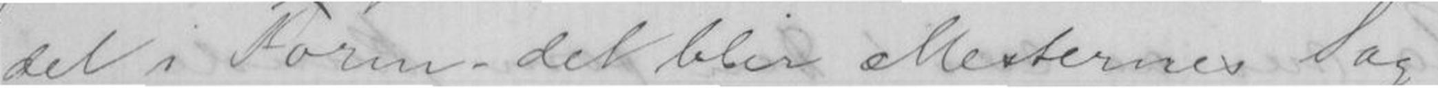det i Form det blir Mesternes Sag
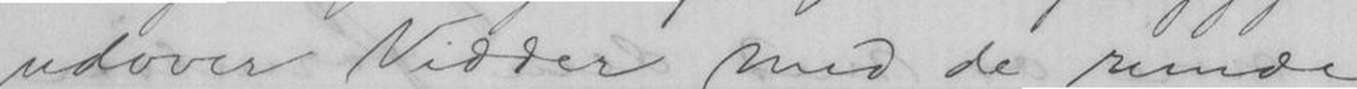udover Vidder med de runde
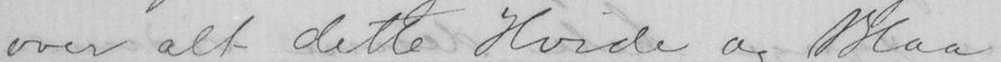over alt dette Hvide og Blaa!
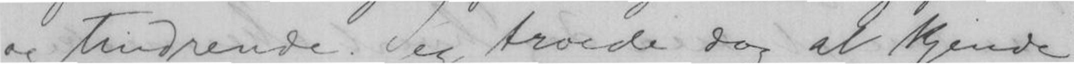og tindrende. Jeg troede dog at kjende
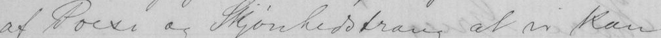af Poesi og Skjønhedstrang at vi kan
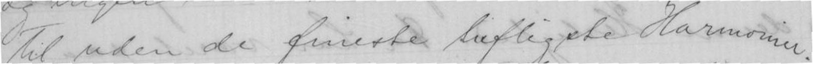til uden de fineste heftigste Harmonier. -
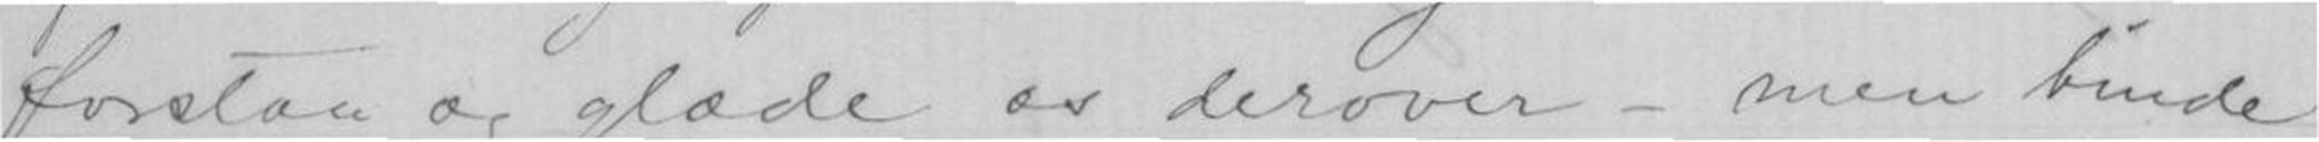forstaa og glæde os derover - men binde
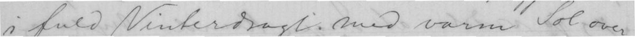i fuld Vinterdragt med varm Sol over
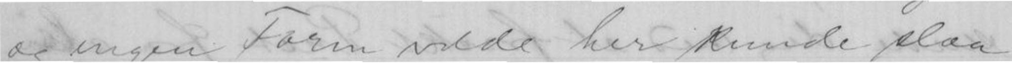og ingen Form vilde her kunde slaa
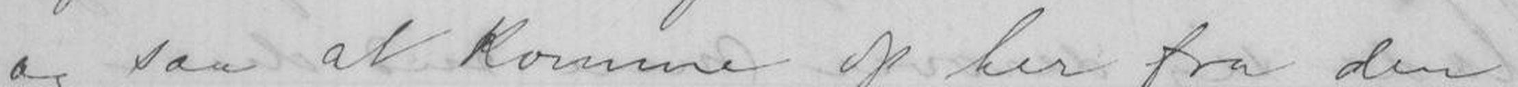og saa at komme op her fra den
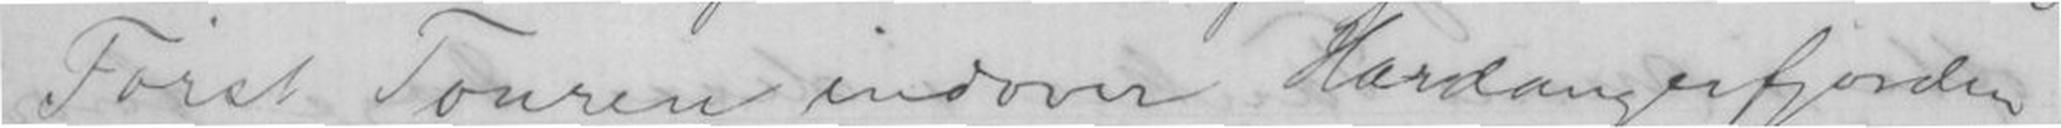Først Touren indover Hardangerfjorden
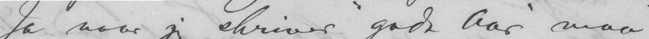Ja naar jeg skriver "godt Aar" maa
